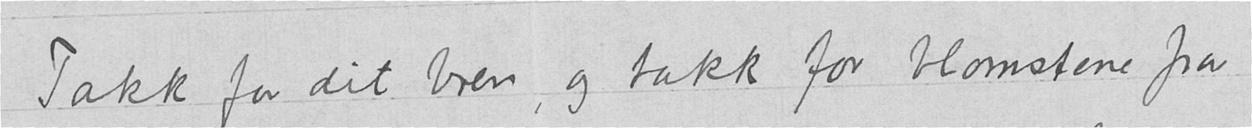Takk for dit brev, og takk for blomstene fra
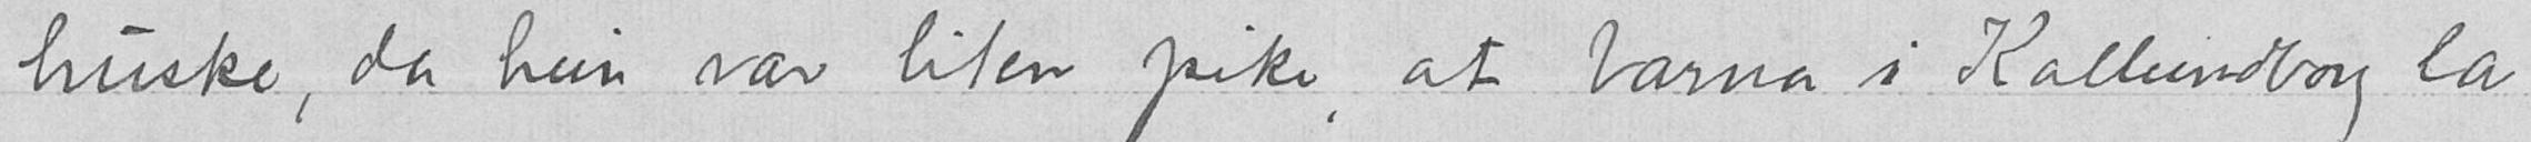huske, da hun var liten pike, at barna i Kallundborg la
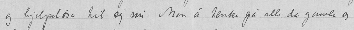og hjelpeløse til sig nu.  Men å tenke pa alle de gamle og
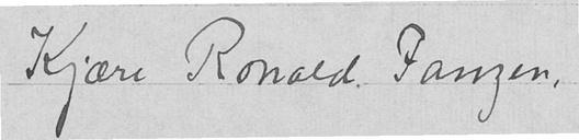Kjære Ronald Fangen,
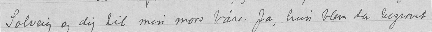Solveig og dig til min mors båre. Ja, hun blev da begravet
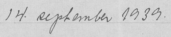14. september 1939.
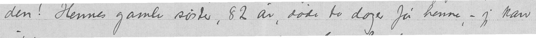den! Hennes gamle søster, 82 år, døde to dager før henne, – jeg kan
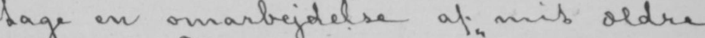tage en omarbejdelse af mit ældre
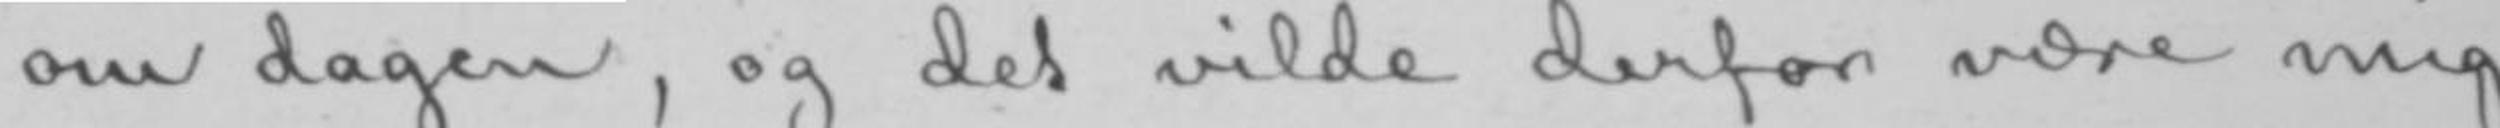om dagen, og det vilde derfor være mig
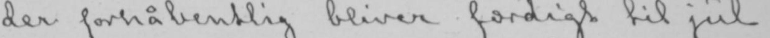der forhåbentlig bliver færdigt til jul.
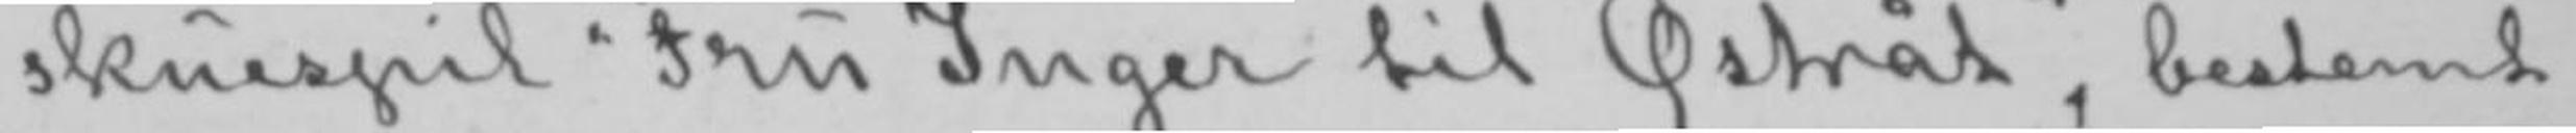skuespil «Fru Inger til Østråt», bestemt
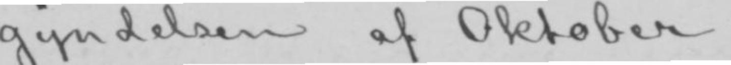gyndelsen af Oktober.
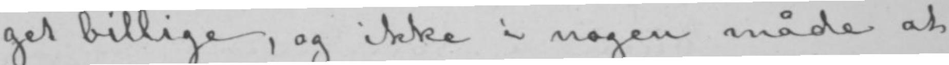get billige, og ikke i nogen måde at
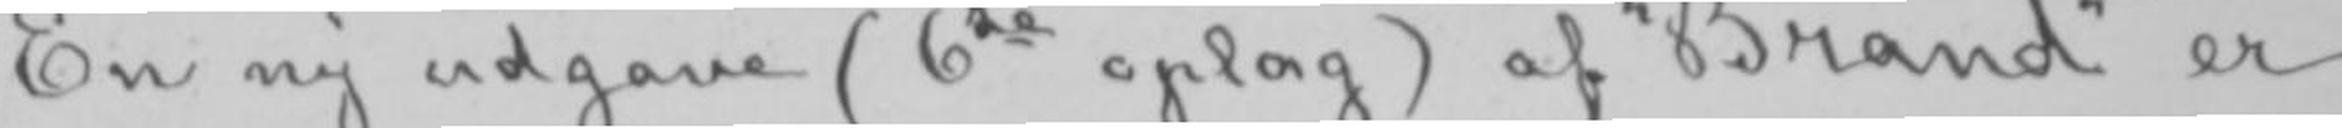En ny udgave (6te oplag) af «Brand» er
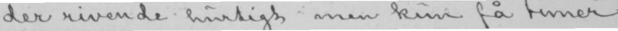der rivende hurtigt men kun få timer
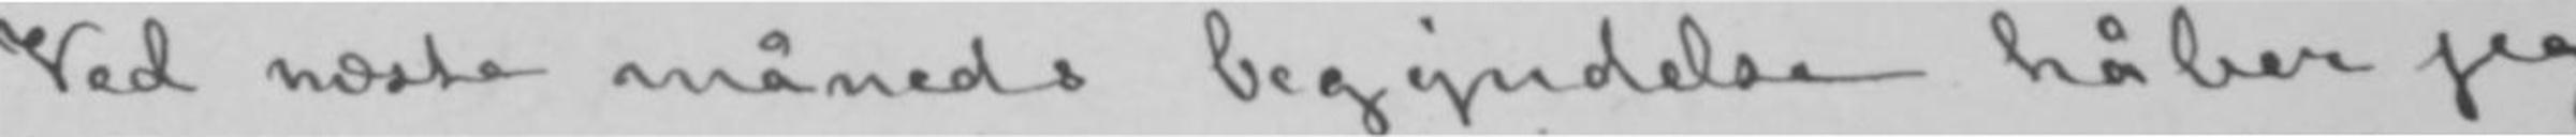Ved næste måneds begyndelse håber jeg
